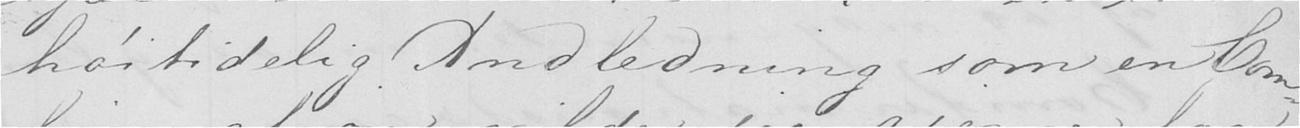høitidelig Andledning som en Com-
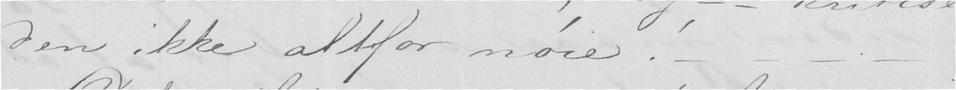den ikke altfor nøie. - - - -
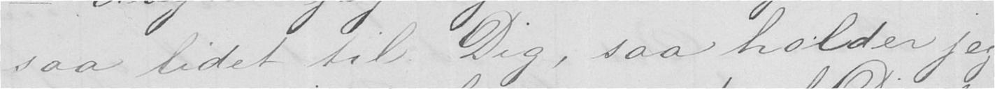saa lidet til Dig, saa holder jeg
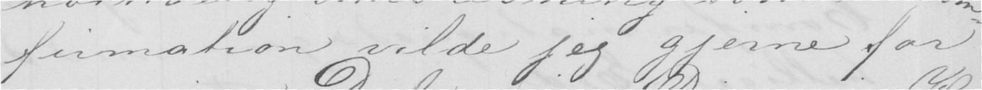firmation vilde jeg gjerne for
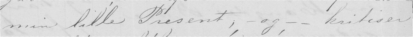min lille Present, - og, - - kritiser
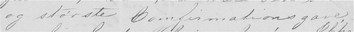og største Comfirmationsgave,
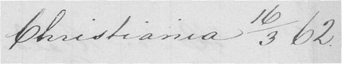Christiania 16/3 62.
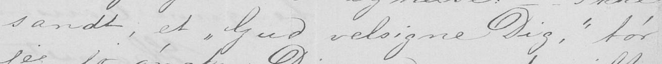sandt, et "Gud velsigne Dig," tør
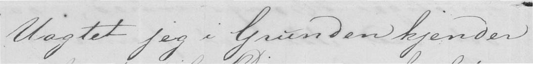Uagtet jeg i Grunden kjender
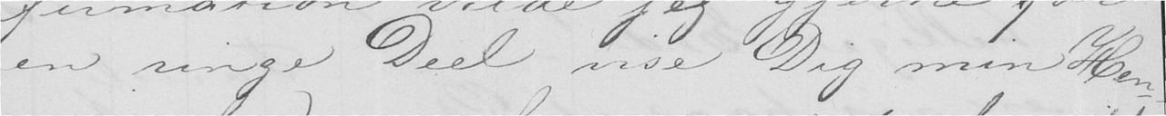en ringe Deel vise Dig min Hen-
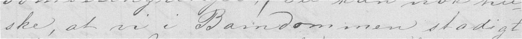ske, at vi i Barndommen stadigt
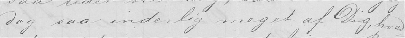dog saa inderlig meget af Dig, hvad
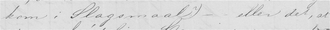kom i Slagsmaal?) - - eller det, at
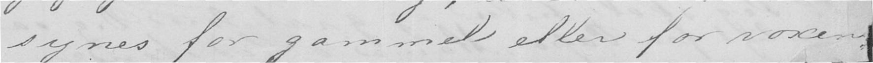synes for gammel eller for voxen.
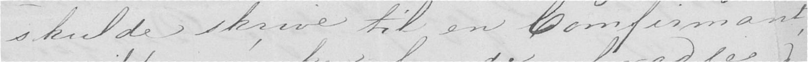skulde skrive til en Comfirmant,
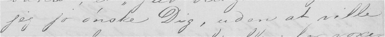jeg jo ønske Dig, uden at ville
